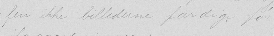fen ikke billederne færdige før
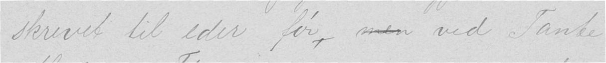skrevet til eder før, men ved Tante
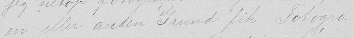en eller anden Grund fik Fotogra-
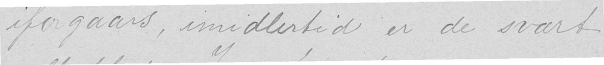iforgaars, imidlertid er de svært
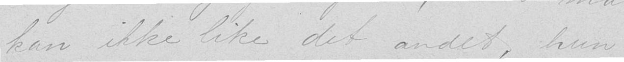kan ikke like det andet, hun
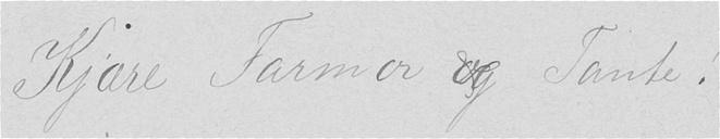Kjære Farmor og Tante!
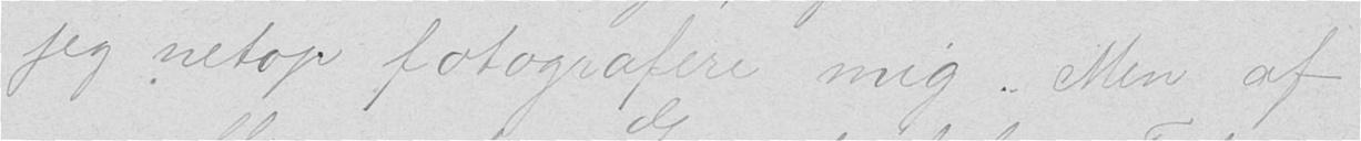jeg netop fotografere mig. Men af
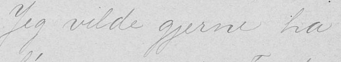Jeg vilde gjerne ha
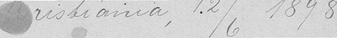Kristiania 12/6 1898
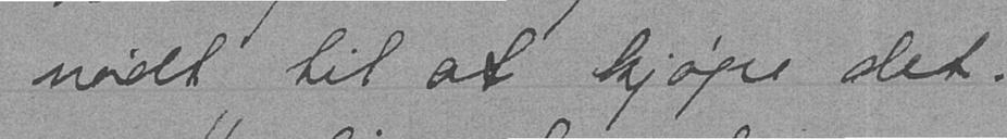nødt til at kjøpe det.
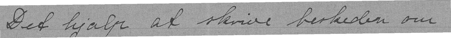Det hjalp at skrive beskeden om
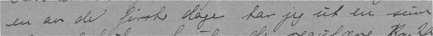en av de første dage tar jeg ut en sum
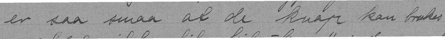er saa smaa at de knap kan brukes
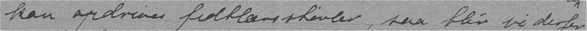kan opdrives feltlærstøvler, saa blir vi derfor
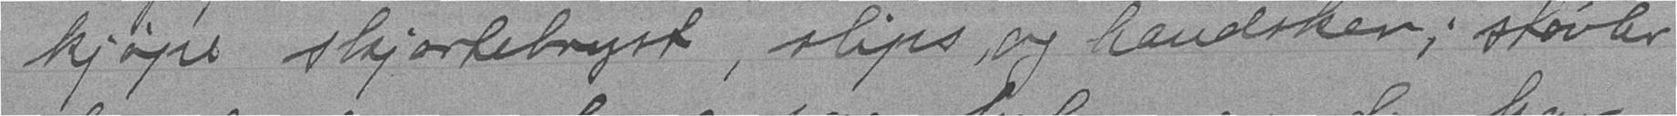kjøpe skjortebryst, slips, og handsker; støvler
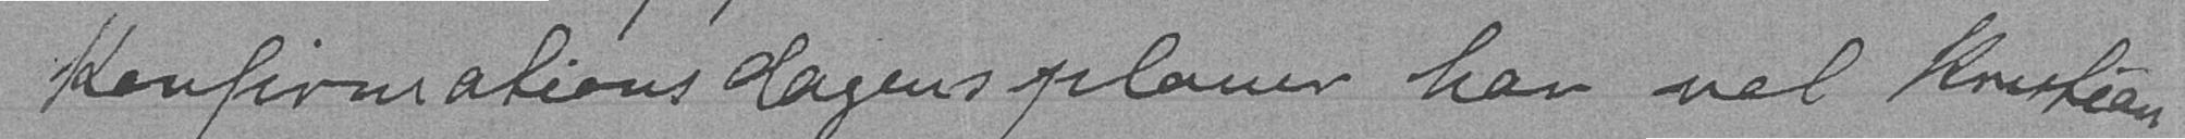Konfirmationsdagens planer har vel Kristian
